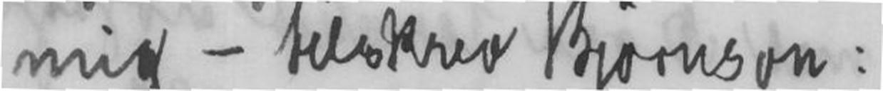mig - tilskrev Bjørnson:
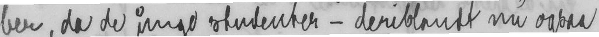ber, da de unge studenter - deriblandt nu ogsaa
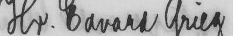Hr. Edvard Grieg!
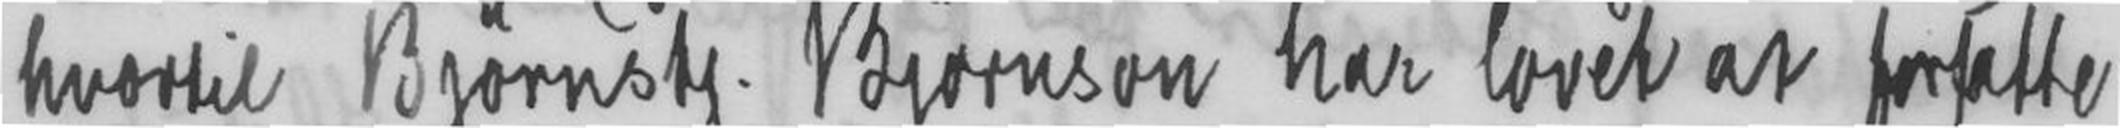hvortil Bjørnstj. Bjørnson har lovet at forfætte
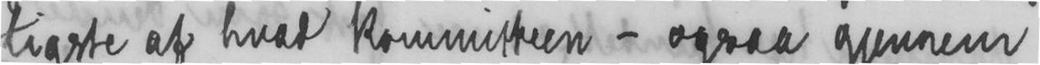tigste af hvad kommitteen - ogsaa gjennem
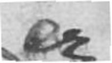er
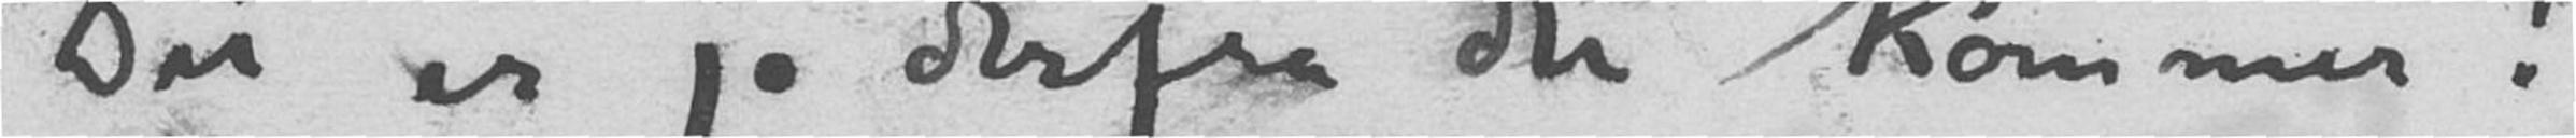Det er jo derfra det kommer!
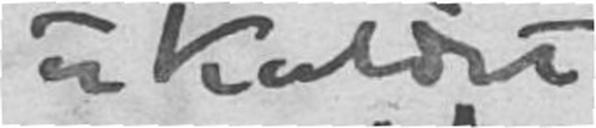ukaldet
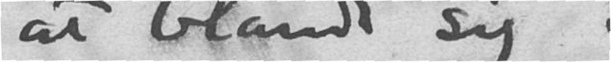at blande sig
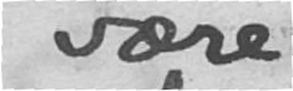være
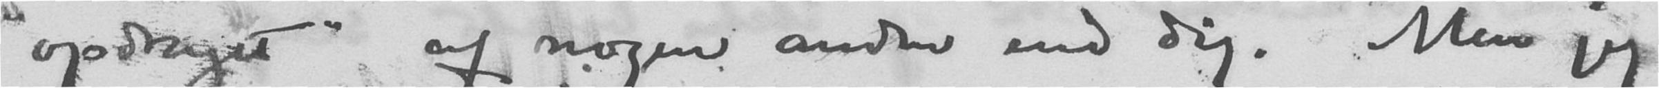"opdraget" af nogen anden end dig. Men jeg
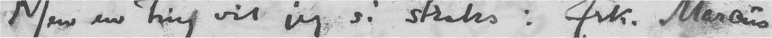Men en ting vil jeg si straks: frk. Marcus
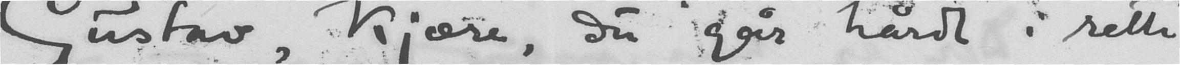Gustav, kjære, du går hårdt i rette
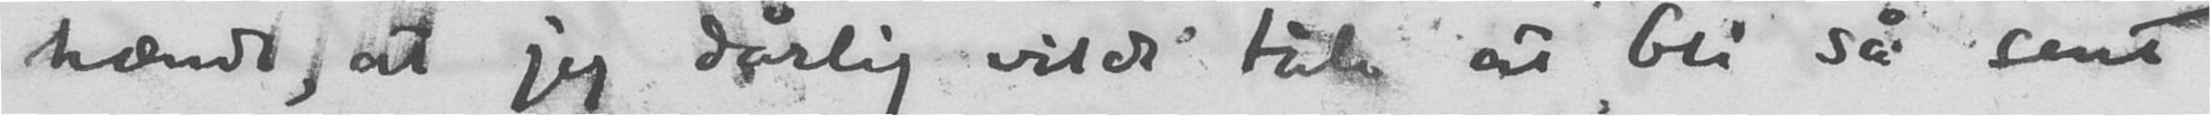hænde, at jeg dårlig vilde tåle at bli så sent
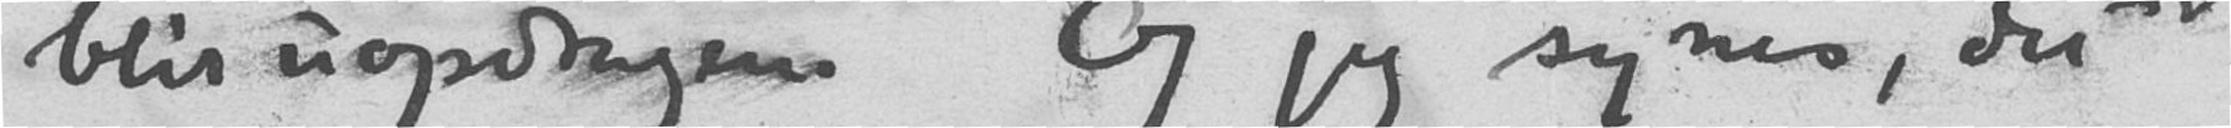blir uopdragen. Og jeg synes, det
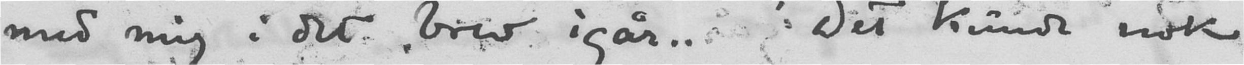med mig i det brev igår.. Det kunde nok
